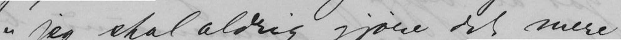"jeg skal aldrig gjøre det mere",
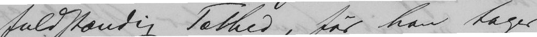fuldstændig Tæthed, før han tager
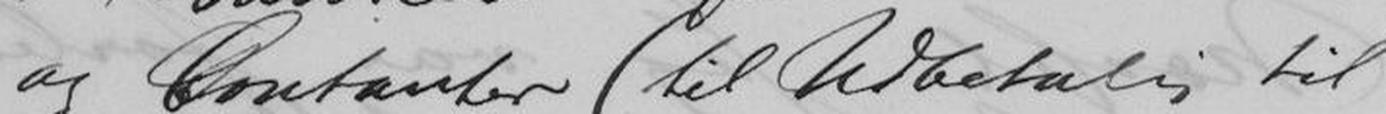og Contanter (til Udbetaling til
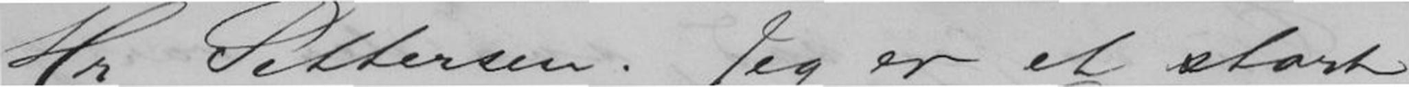Hr Pettersen. Jeg er et stort
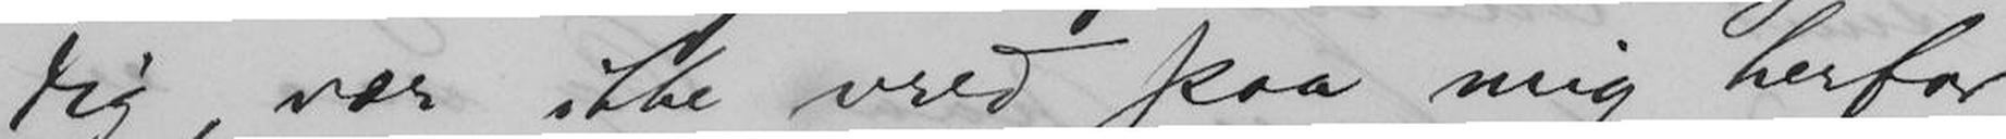Dig, vær ikke vred paa mig herfor
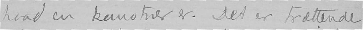hvad en kunstner er. Det er trættende
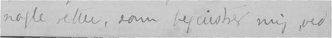nogle retter, som begeistrer mig, ved
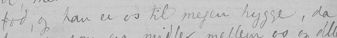fod, og han er os til megen hygge, da
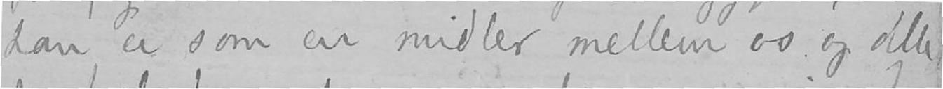han er som en midler mellem os og alle
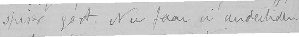spiser godt. Nu faar vi undertiden
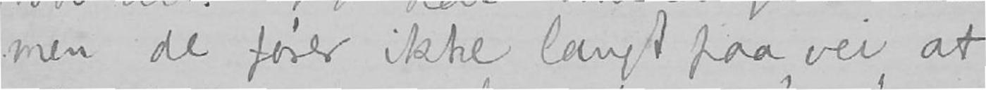men de fører ikke langt paa vei at
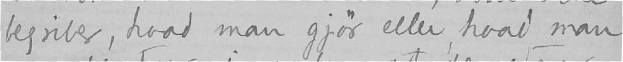begriber, hvad man gjør eller hvad man
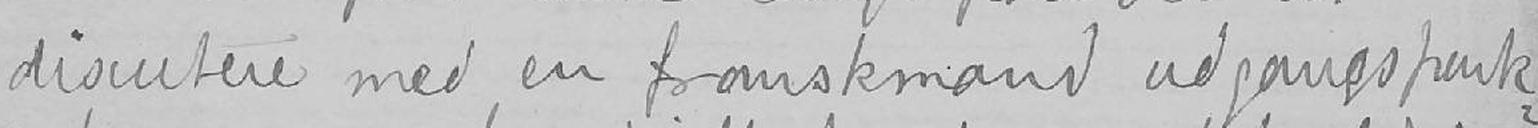discutere med en franskmand udgangspunk-
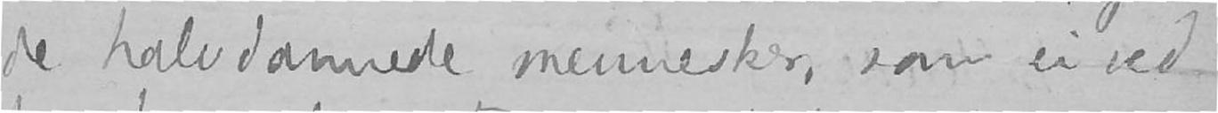de halvdannede mennesker, som ei ved
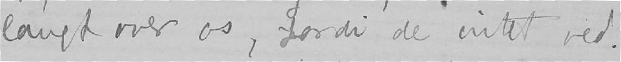langt over os, fordi de intet ved.
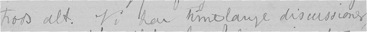trods alt. Vi har timelange discussioner,
